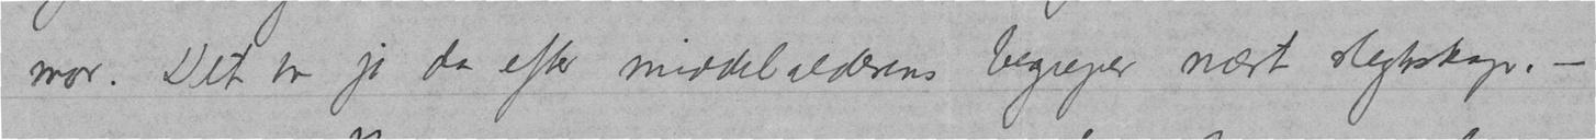mor. Det var jo da efter middelalderens begreper nært slegtskap. –
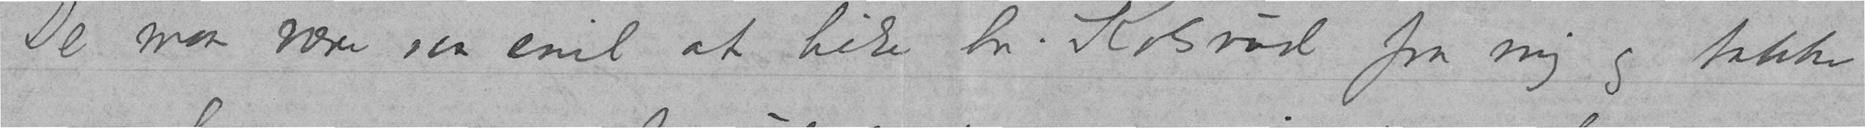De maa være saa snil at hilse hr. Kolsrud fra mig og takke
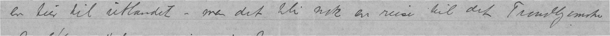en tur til utlandet – men det blir nok en reise til det Trondhjemske
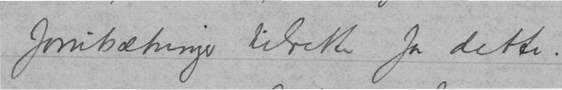forutsætninger tilrette for dette.
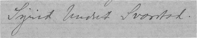Sigrid Undset Svarstad.
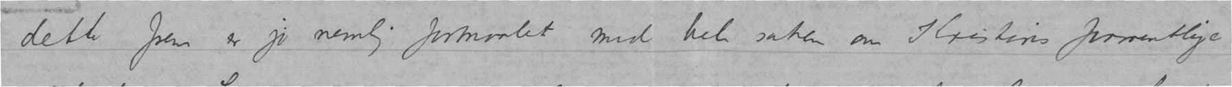Dette frem er jo nemlig formaalet med hele saken om Kristins formentlige
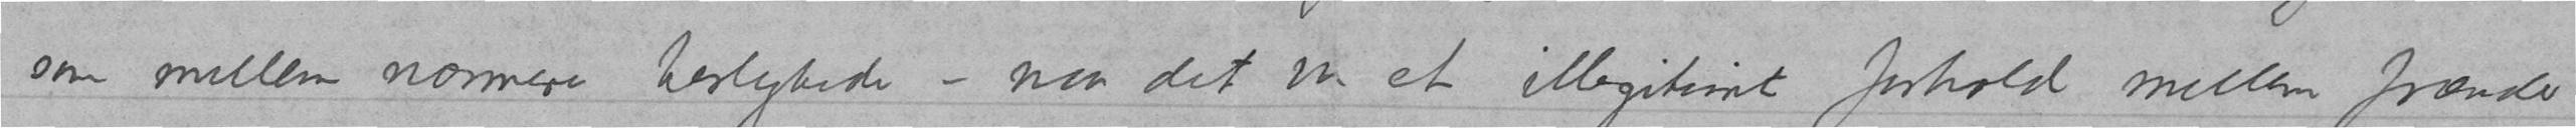som mellem nærmere beslegtede – naar det var et illegitimt forhold mellem frænder
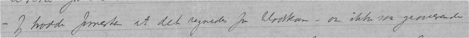– Jeg trodde forresten at det regnedes for blodskam – om ikke saa graverende
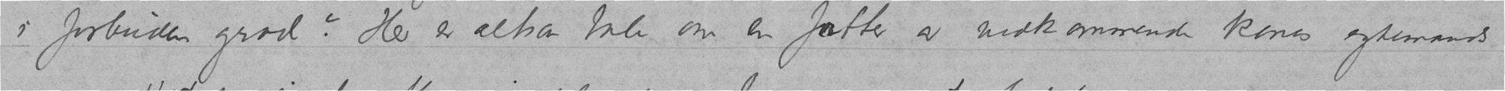i forbuden grad? Her er altsaa tale om en fætter av vedkommende kones ægtemands
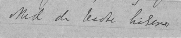Med de bedste hilsener
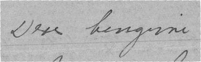Dere hengivne
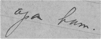ogsaa ham.
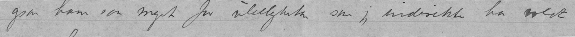ogsaa ham saa meget for ulelligheten som jeg indirekte har voldt
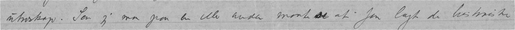utroskap. Saa jeg maa paa en eller anden maate se at faa lagt de historiske
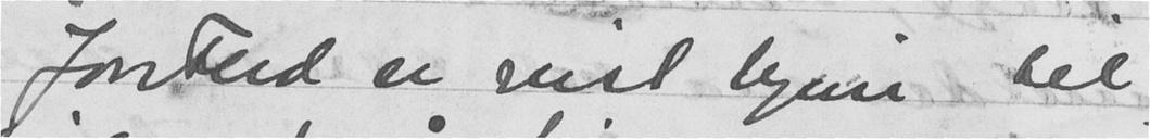Jonfrid er reist hjem til
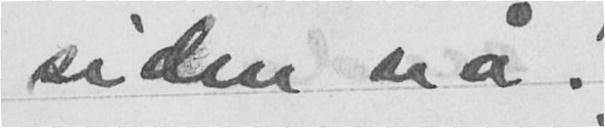siden nå!
